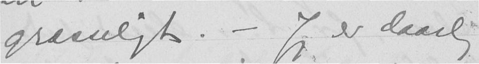græsseligt. - Jeg er daarlig
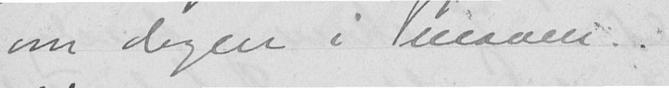om dagen i maven.
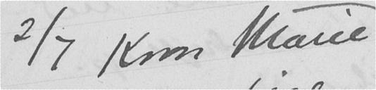2/7 Kom Marie
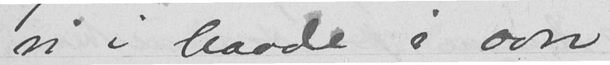vi i baade i ovn
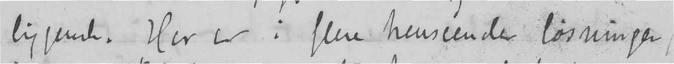liggende. Her er i flere henseender løsninger
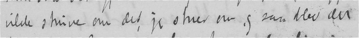vilde skrive om det, jeg skrev om, og saa blev det
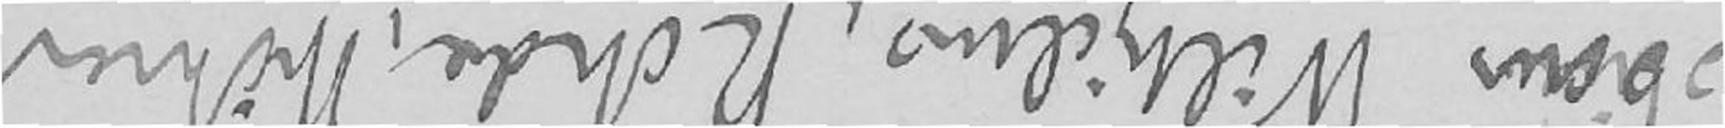sons, Willumsen, Rohde, Möhner,
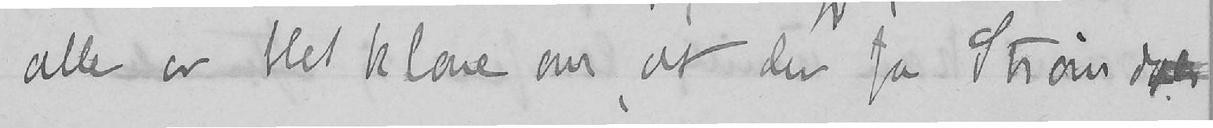alle er blit klare om, at der fra Strømdals
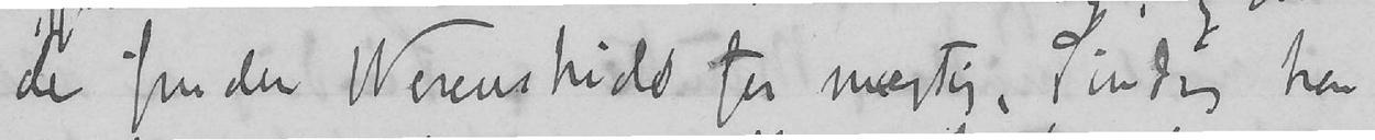de finder Werenskiold for mægtig, Sinding har
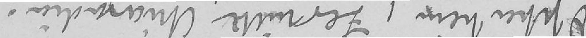Oppenheim, Zerritte, Chiaradia.
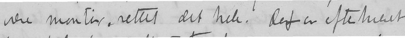være montør, rettet det hele. Det er efterhvert
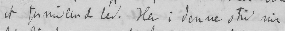et formilende led. Her i denne strid nu
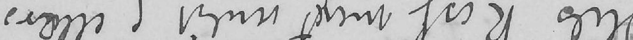Hils Ross meget venligt og ellers
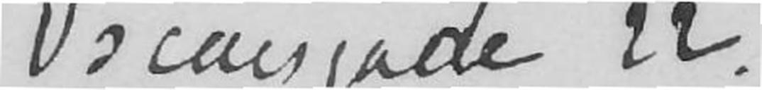Oscarsgade 22.
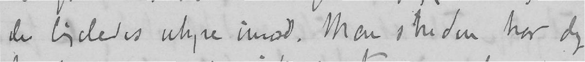de ligeledes uhyre imod. Men striden har dog
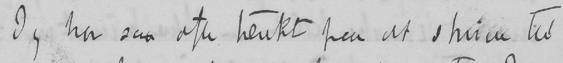Jeg har saa ofte tænkt paa at skrive til
